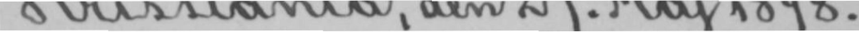Kristiania, den 29. Maj 1898.
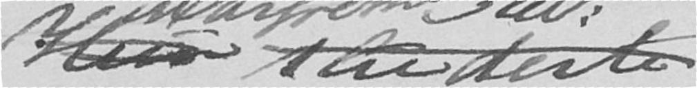Hun studerte
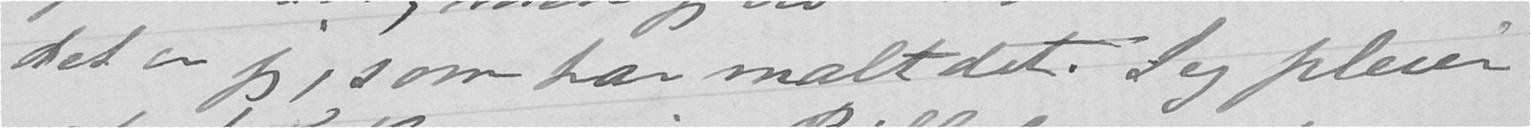det er jeg, som har malt det. Jeg pleier
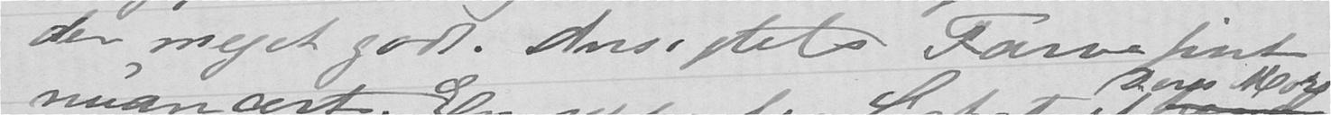nuancert. En ypperlig Lighet ifra hendes
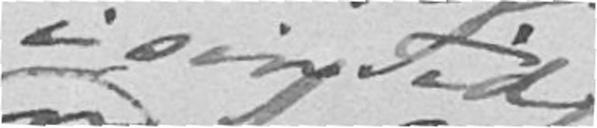i sin Tid
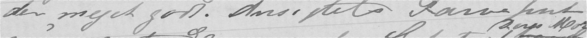der meget godt. Ansigtets Farve fint
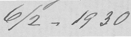6/2 - 1930
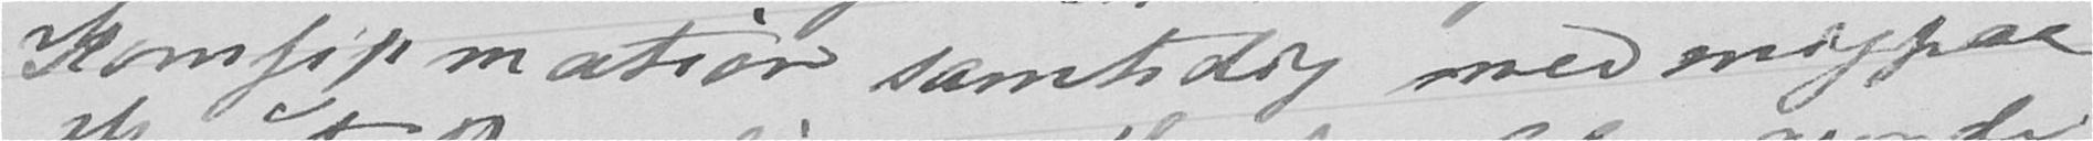Komfirmation samtidig med meg paa
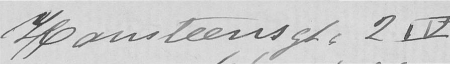Hansteensgt. 2 IV
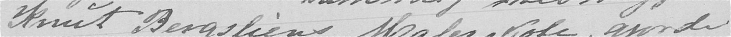Knut Bergstiens Malerskole, gjorde
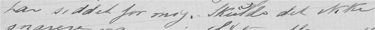har siddet for mig. Skulde det ikke
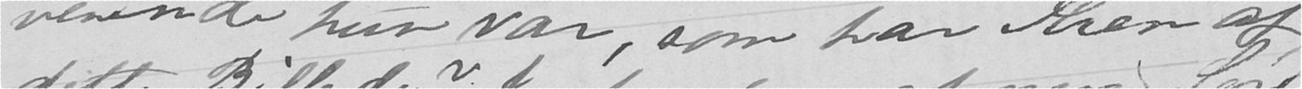veninde hun var, som har Æren af
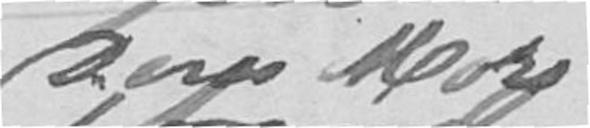Dage Mors
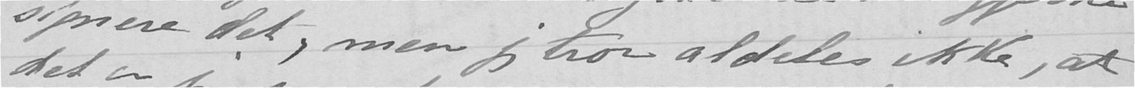signere det, men jeg tror aldeles ikke, at
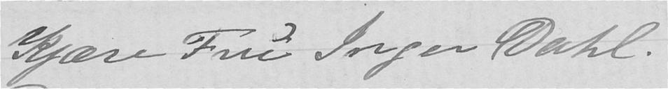Kjære Fru Inger Dahl.
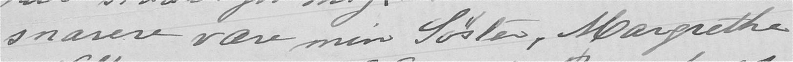snarere være min Søster, Margrethe
